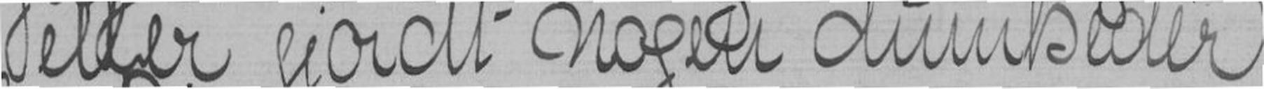eller gjordt noget dumheder
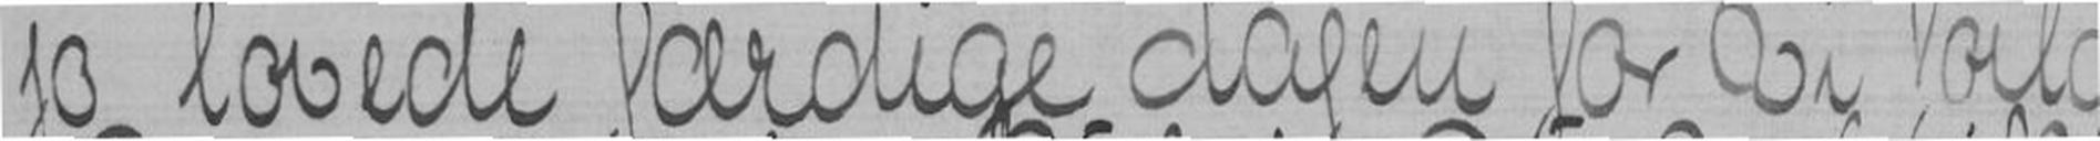jo lovede færdige dagen før vi forlod
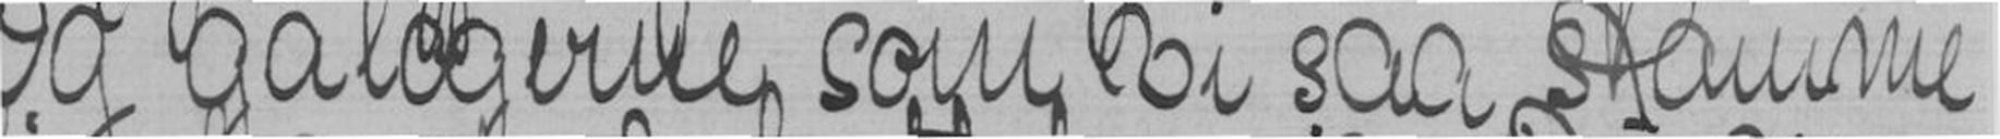Og galogerne som vi saa skamme
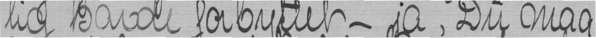lig havde forbyttet - ja, Du maa
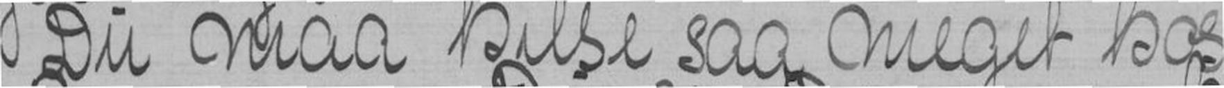Du maa hilse saa meget hos
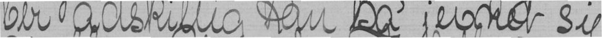ber adskillig kan ha'jevnet sig
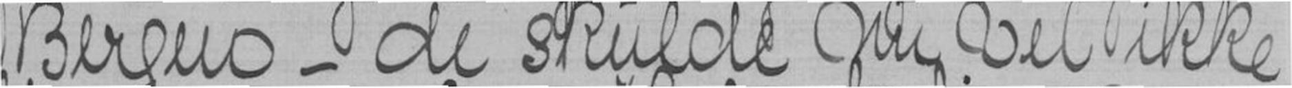Bergen - de skulde nu vel ikke
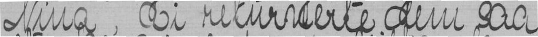Nina, vi returnerte dem saa
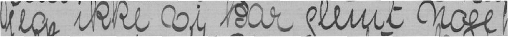jeg ikke vi har glemt noget
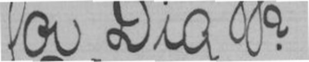for Dig? -
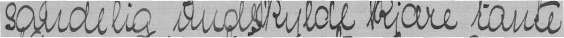sandelig undskylde kjære tante
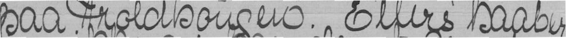paa Troldhougen. Ellers haaber
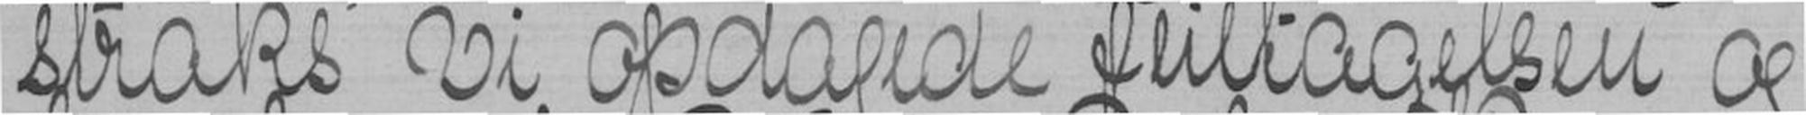straks vi opdagede feiltagelsen og
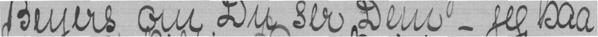Beyers om Du ser Dem - jeg haa-
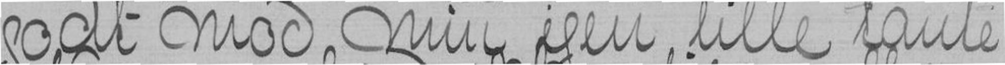godt mod min egen lille tante
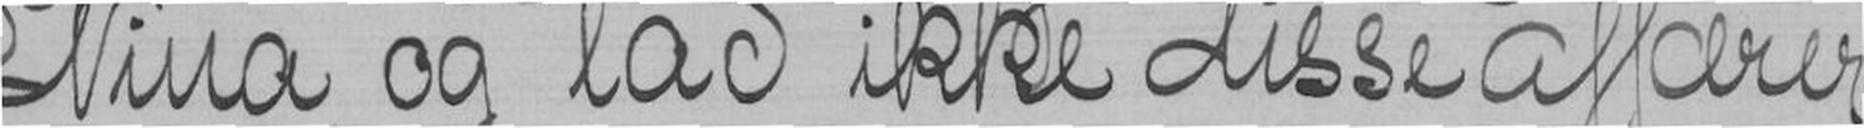Nina og lad ikke disse affærer
